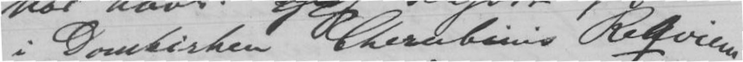i Domkirken Cherubinis Reqviem.
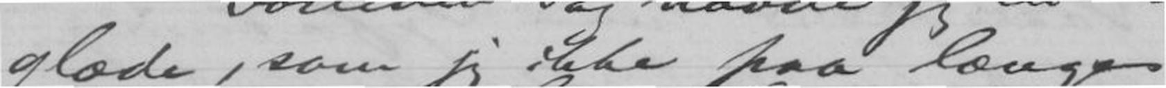glæde, som jeg ikke paa længe
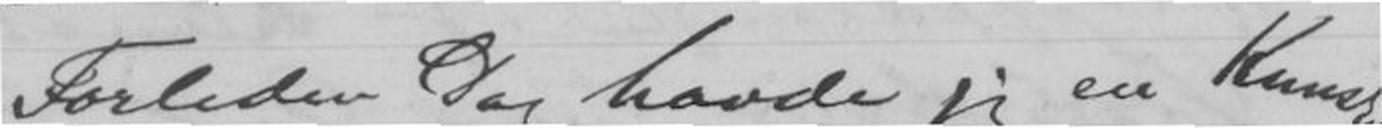Forleden Dag havde jeg en Kunst-
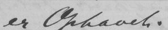er Ophavet.
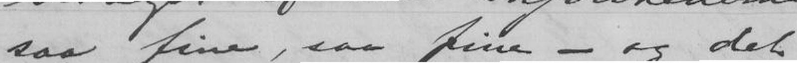saa fine, saa fine - og det
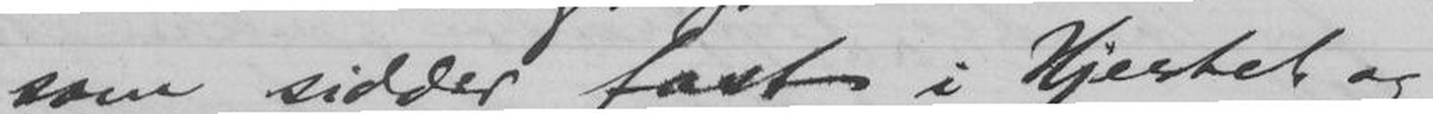som sidder fast i Hjertet og
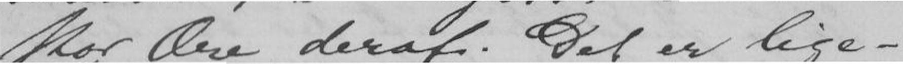stor Ære deraf. Det er lige-
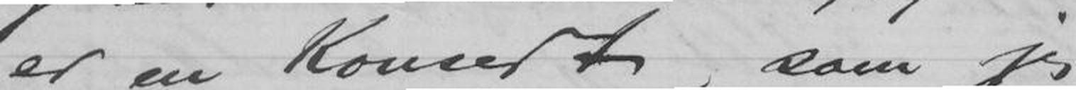er en Konsert, som jeg
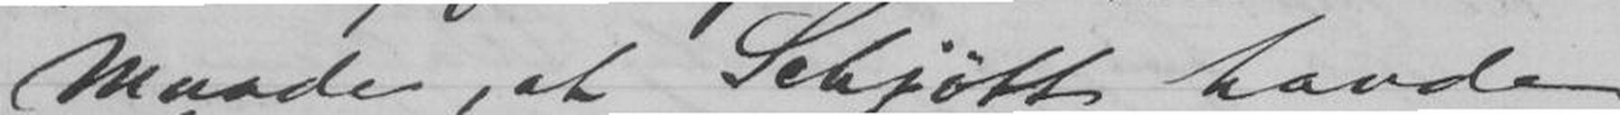Maade, at Schjøtt havde
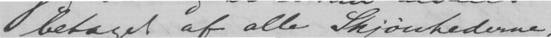betaget af alle Skjønhederne,
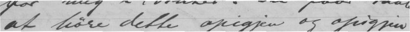at høre dette opigjen og opigjen
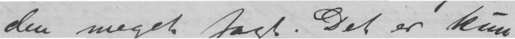den meget sagt. Det er kun
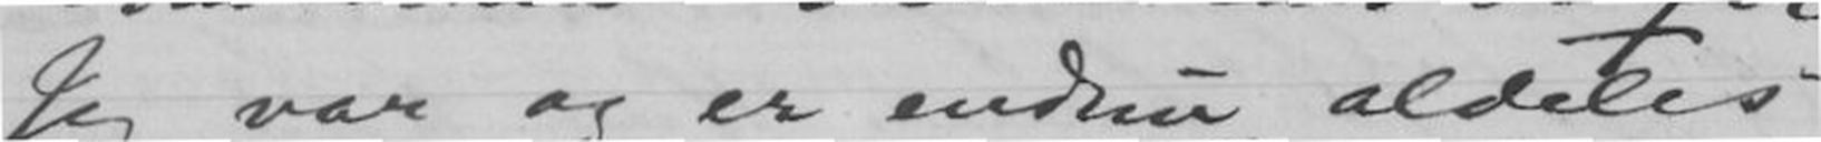Jeg var og er endnu aldeles
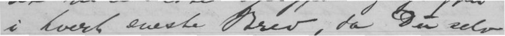i hvert eneste Brev, da Du selv
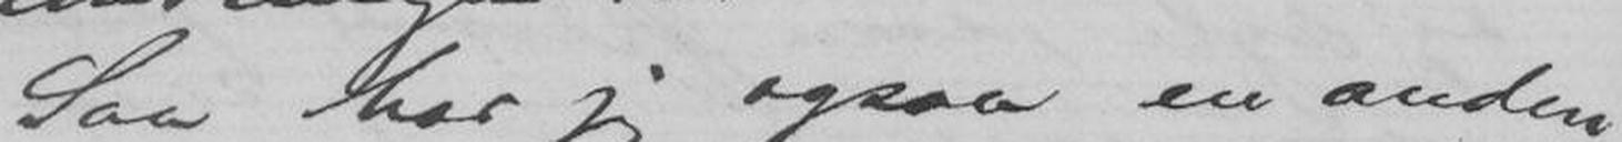Saa har jeg ogsaa en anden
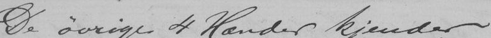De øvrige 4 Hænder kjender
